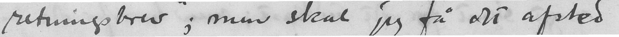retningsbrev"; men skal jeg få det afsted
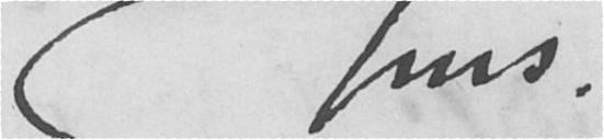Jens
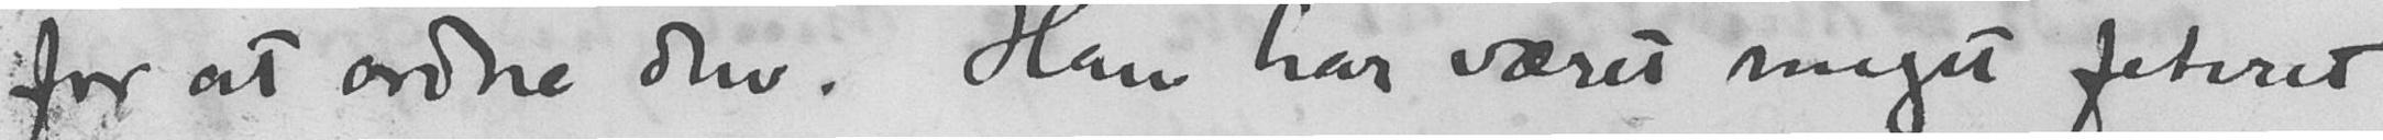for at ordne den. Han har været meget feteret
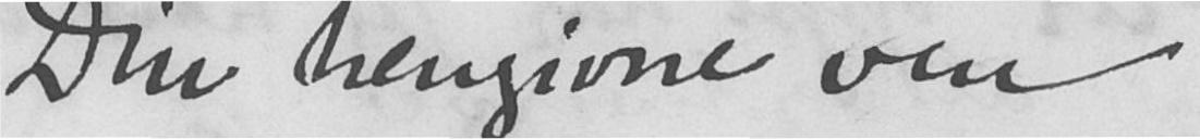Din hengivne ven
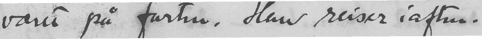været på farten. Han reiser iaften.
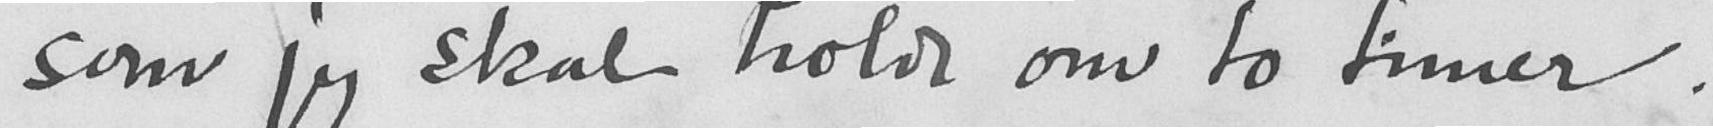som jeg skal holde om to timer.
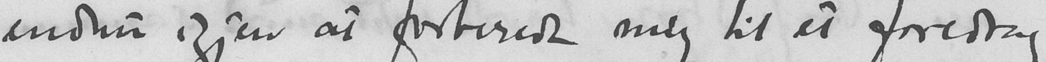endnu igjen at forberede mig til et foredrag
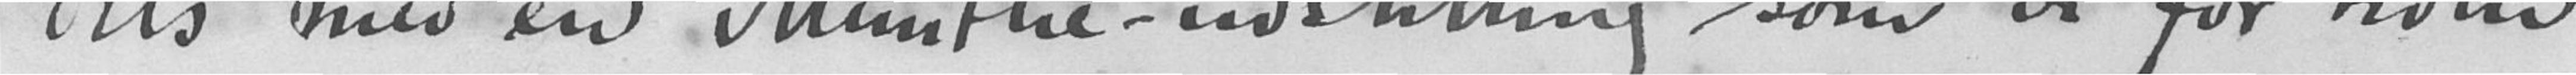dels med en Munthe-udstilling som vi for tiden
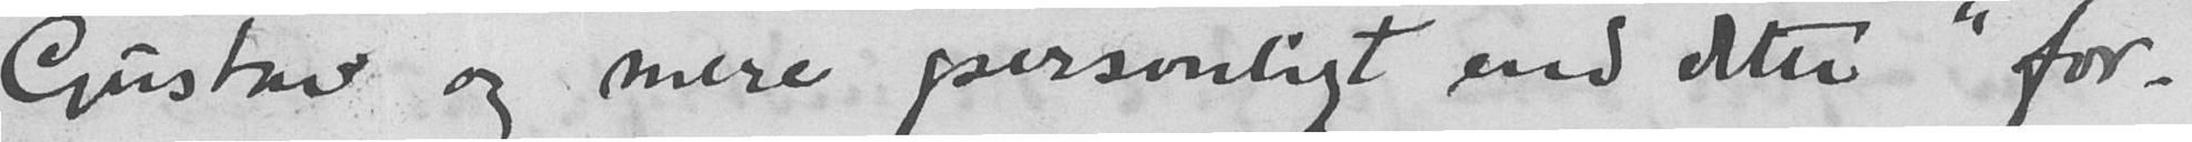Gustav og mere personligt end dette " for-
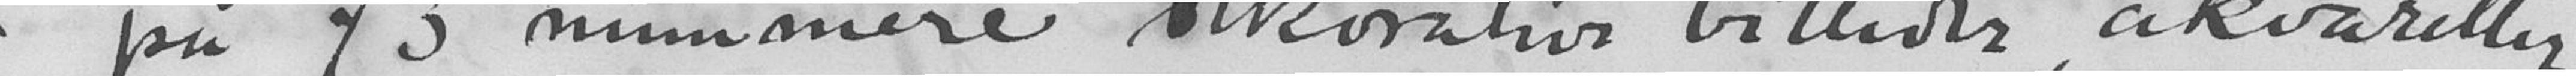på 73 nummere dekorative billeder, akvareller
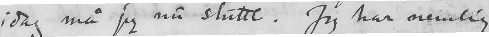idag må jeg nu slutte. Jeg har nemlig
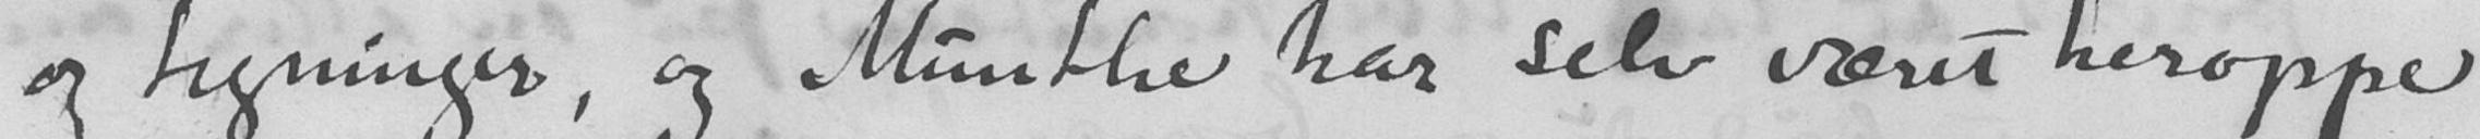og tegninger, og Munthe har selv været heroppe
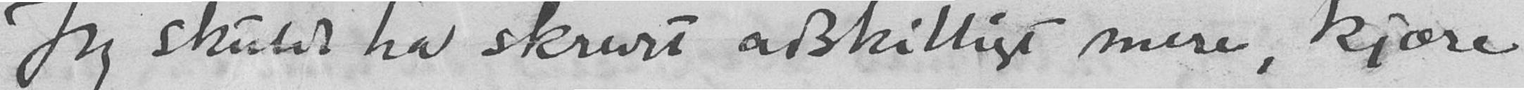Jeg skulde ha skrevet adskilligt mere, kjære
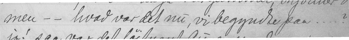men - - hvad var det nu, vi begyndte paa....?
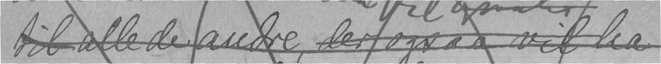til alle de andre, der ogsaa vil ha
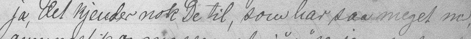ja, det kjender nok De til, som har saa meget m
